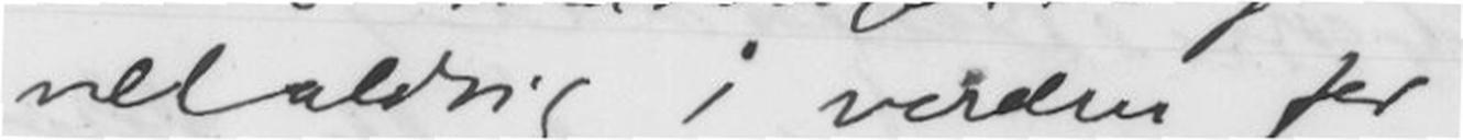vel aldrig i verden for
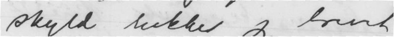skyld lukker jeg brevet
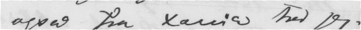også fra Xania tror jeg.
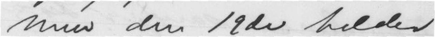Men den 19de holder
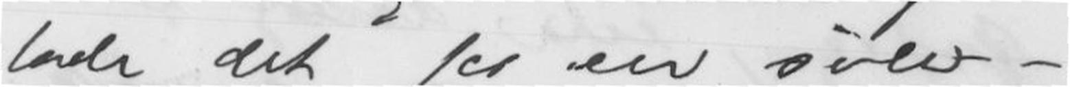lade det for en sølv-
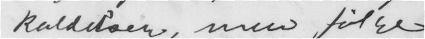kaldetser, men følge
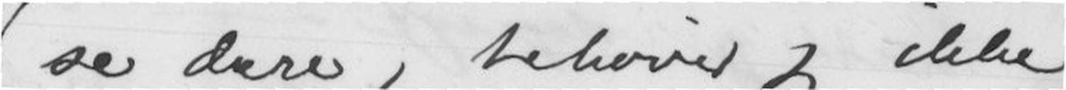se dere, behøver jeg ikke
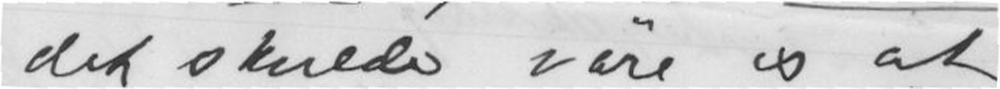det skulde väre og at
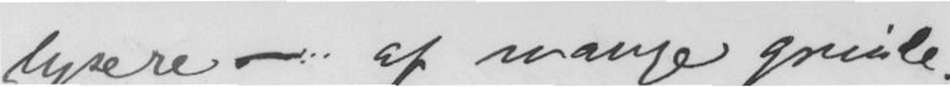lysere - af mange grunde.
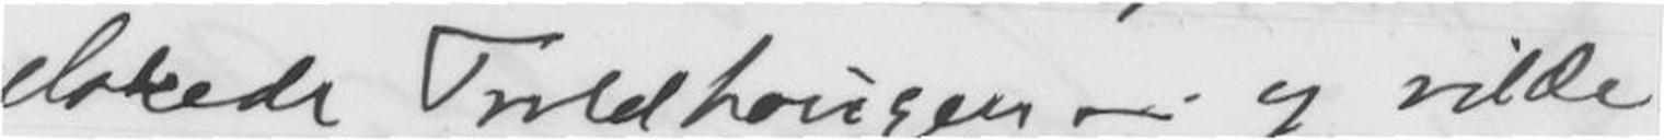elskede Troldhougen - og vilde
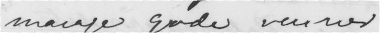mange gode venner
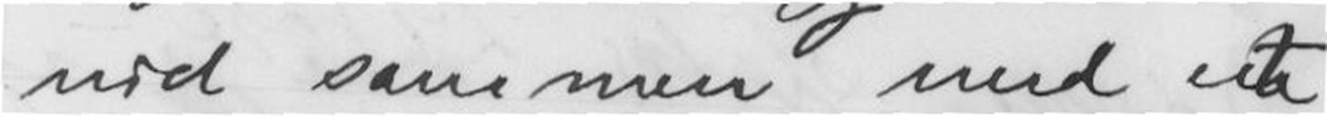ned sammen med et
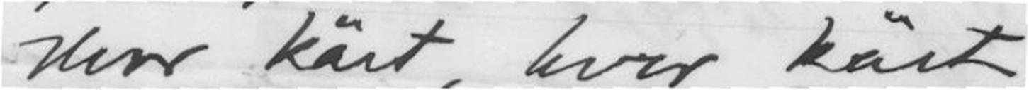Hvor kärt, hvor kärt
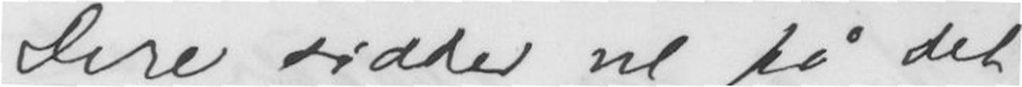-Dere sidder vel på det
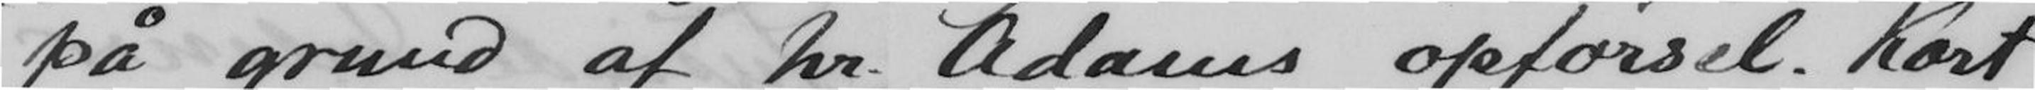på grund af hr. Adams opførsel. Kort
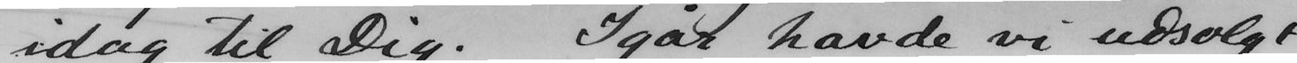idag til Dig. Igår havde vi udsolgt
